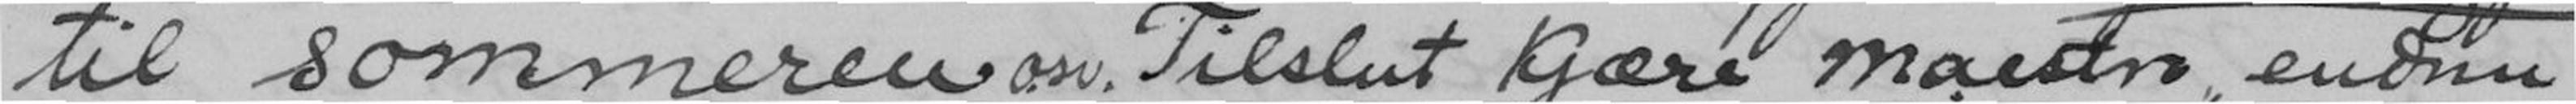til sommeren o.s.v. Tilslut kjære Maestro, endnu
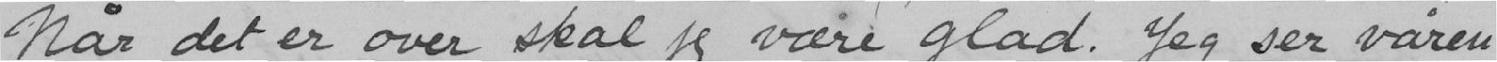Når det er over skal jeg være glad. Jeg ser våren
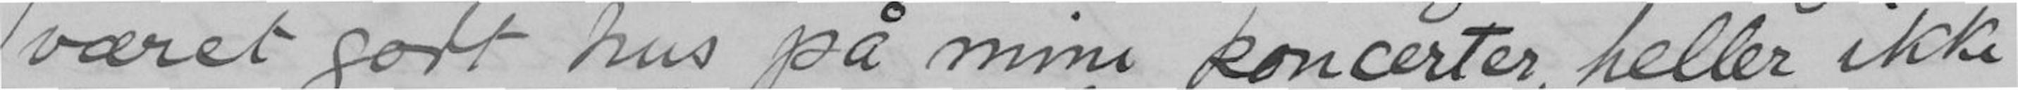været godt hus på mine koncerter, heller ikke
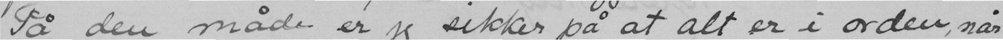På den måde er jeg sikker på at alt er i orden, når
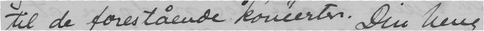til de forestående koncerter. Din heng.
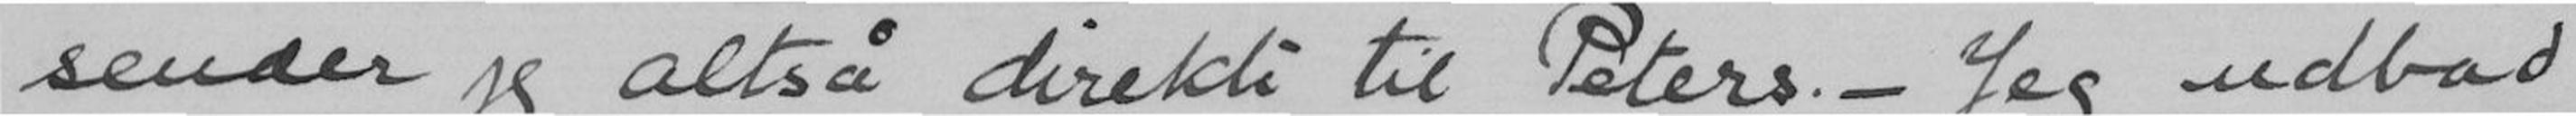sender jeg altså direkte til Peters. - Jeg udbad
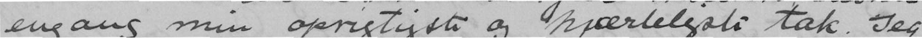engang min oprigtigste og hjærteligste tak. Jeg
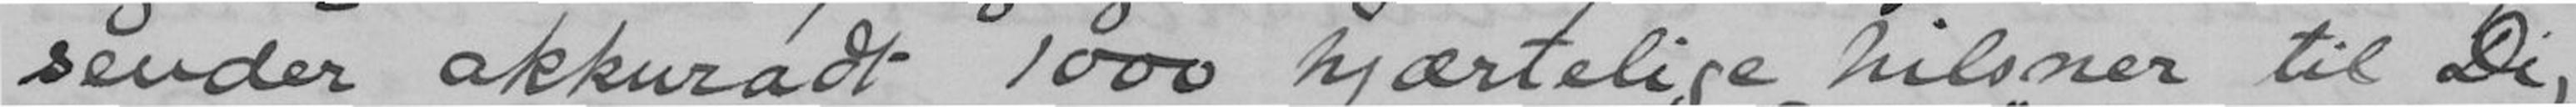sender akkuradt 1000 hjærtelige hilsner til Dig
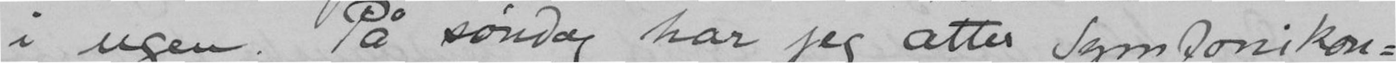i ugen. På søndag har jeg atter Symfonikon-
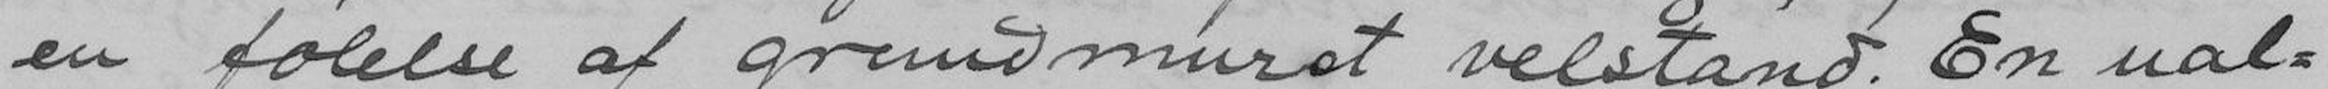en følelse af grundmuret velstand. En ual-
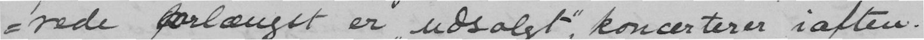rede forlængst er udsolgt, koncerterer iaften.
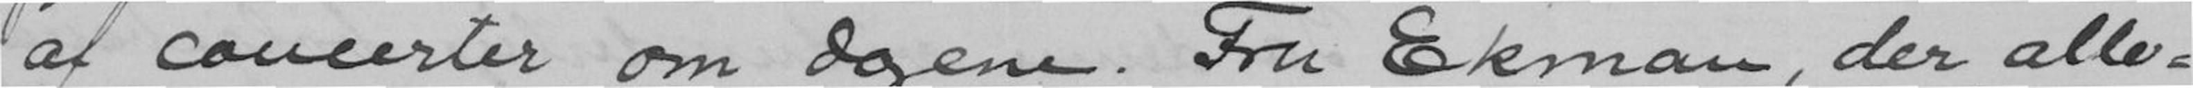af concerter om dagene. Fru Ekman, der alle-
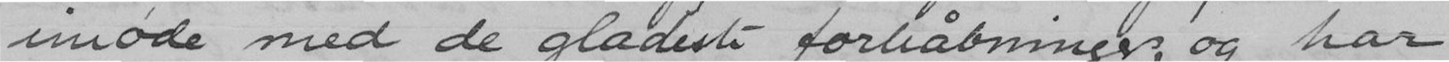imøde med de gladeste forhåbninger, og har
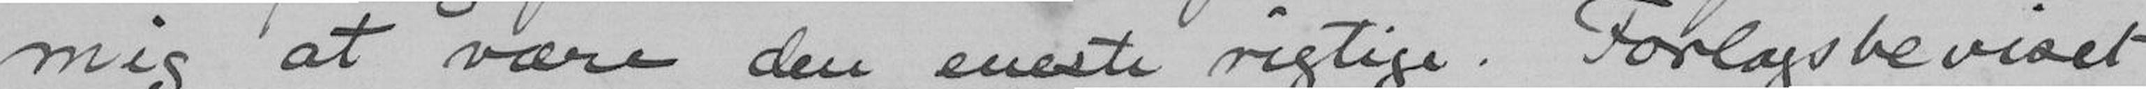mig at være den eneste rigtige. Forlagsbeviset
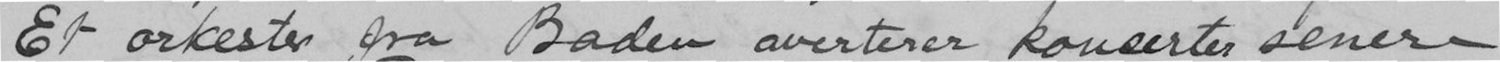Et orkester fra Baden averterer konserter senere
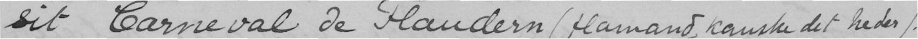sit Carneval de Flandern (flamand, kanske det heder).
